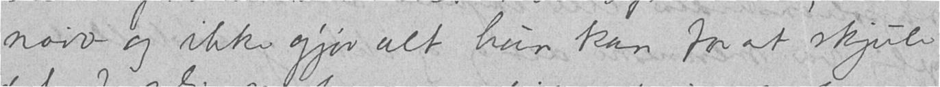naiv og ikke gjør alt hun kan for at skjule
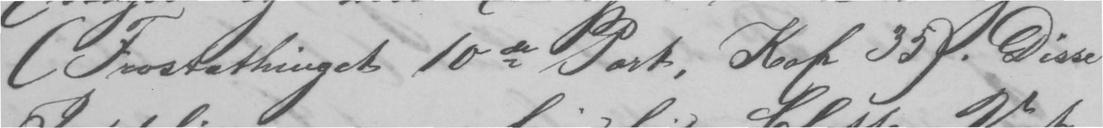(Frostathinget 10de Part, Kap 35). Disse
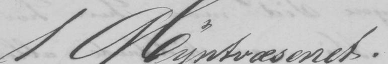I Myntvæsenet.
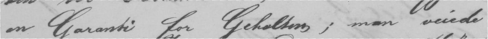en Garanti for Gehalten; man veiede
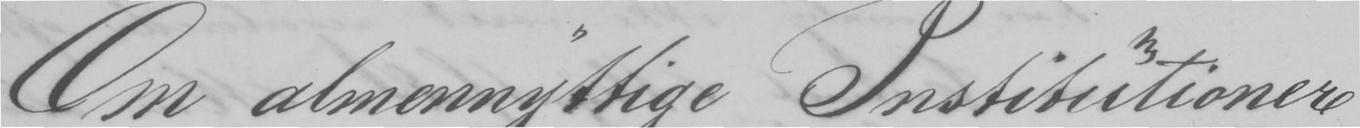Om almennyttige Institutioner
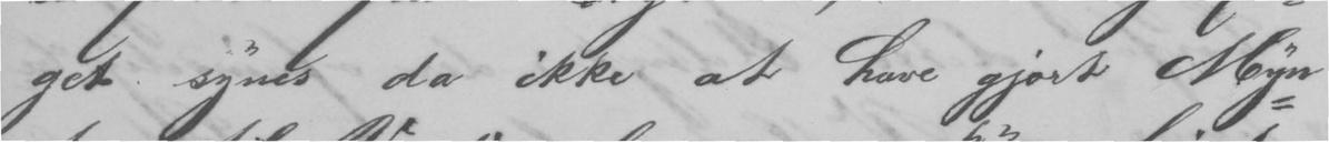get synes da ikke at have gjort Myn-
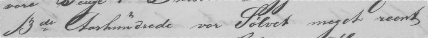13de Aarhundrede var Sølvet meget reent
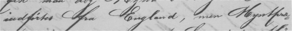indførtes fra England, men Myntpræ-
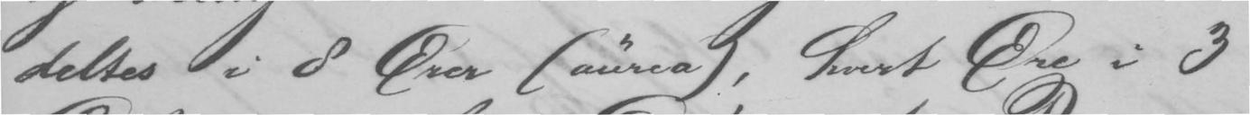deltes i 8 Ører (aurea), hvert Øre i 3
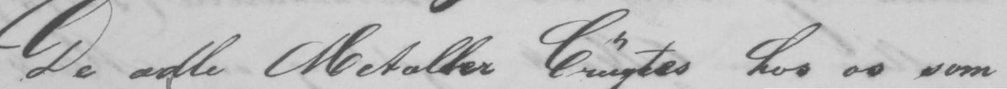De ædle Metaller Brugtes hos os som
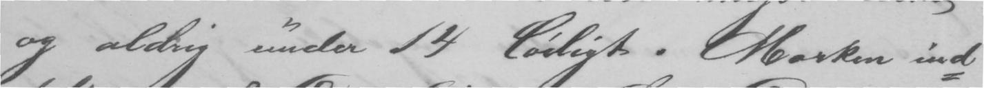og aldrig under 14 loiligt. Marken ind-
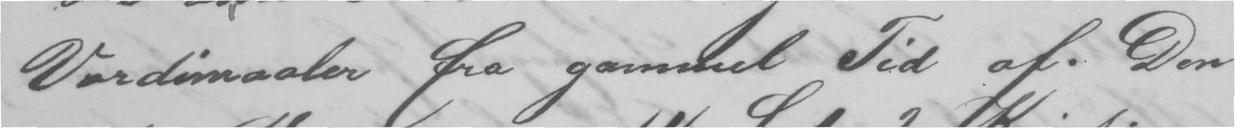Værdimaaler fra gammel Tid af. Den
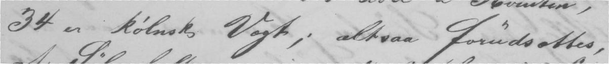34 er kølnsk Vægt; altsaa forudsættes,
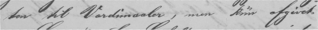ten til Værdimaaler, men kun afgivet
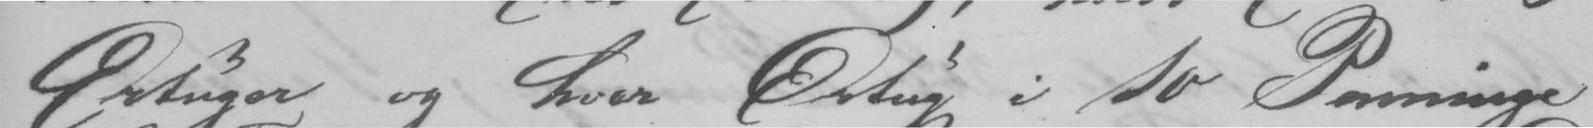Ørtuger og hver Ørtug i to Penninge
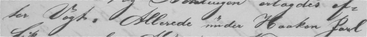ter Vægt. Allerede under Haakon Parl
